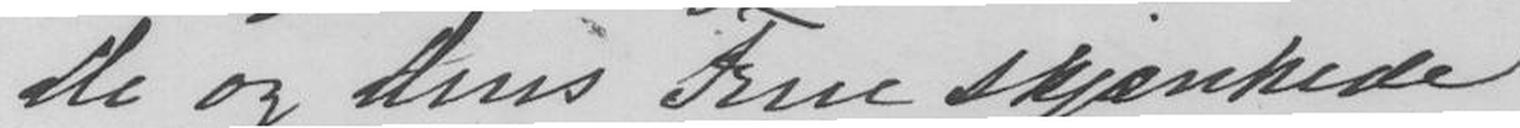De og Deres Frue skjænkede
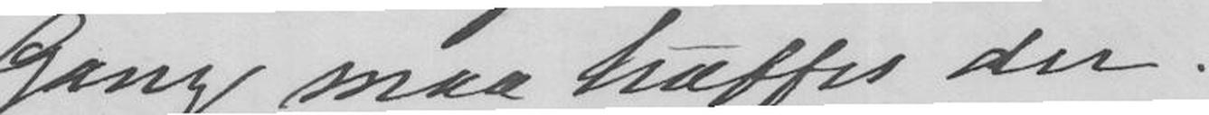Gang maa træffes der.
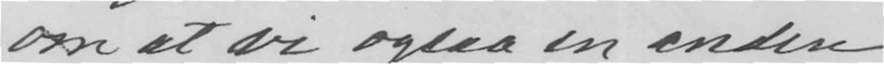om at vi ogsaa en anden
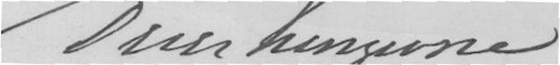Deres hengivne
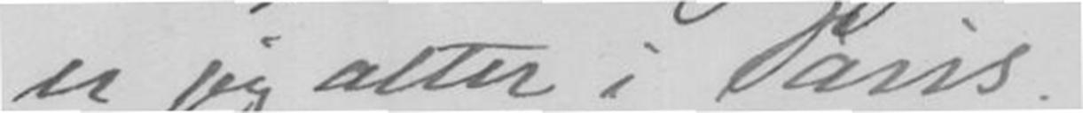er jeg atter i Paris.
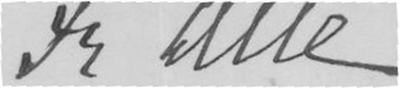Fr Due
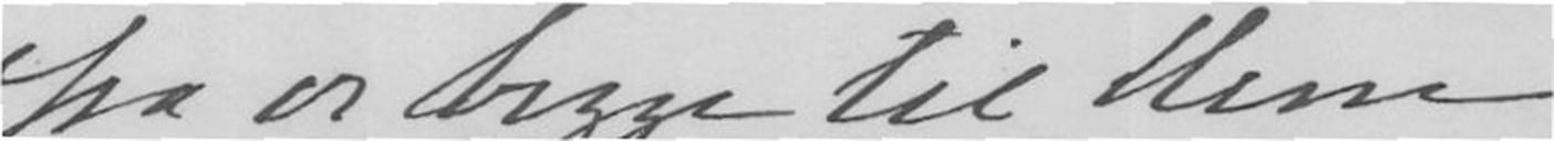fra os begge til Dem
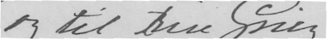og til Fru Grieg
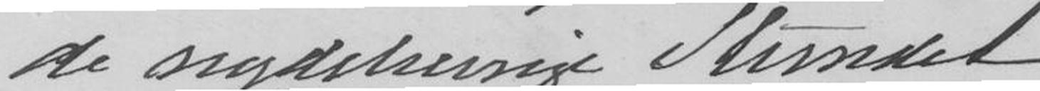de nydelsesrige Stunde
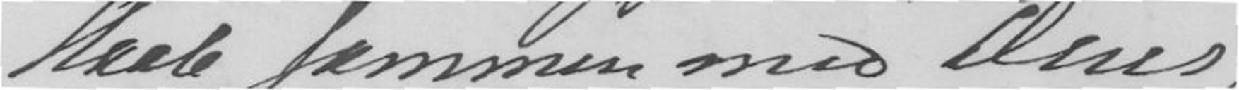Haab sammen med Deres,
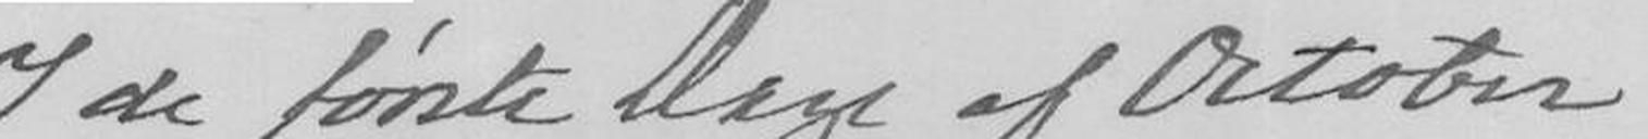I de første Dage af October
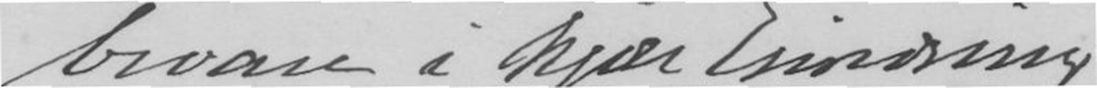bevare i kjær Erindring
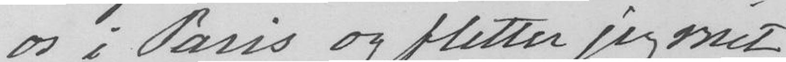os i Paris og fletter jeg mit
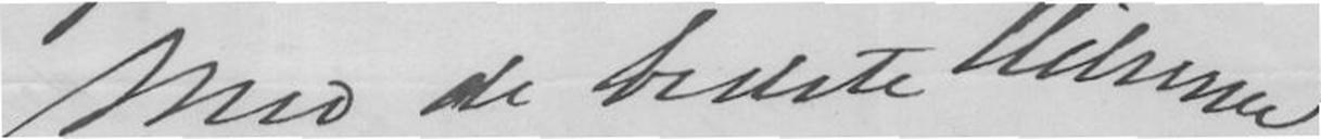Med de bedste Hilsener
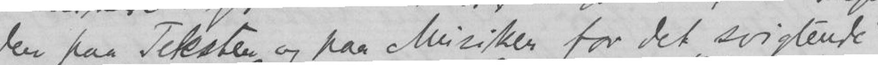den paa Teksten og paa Musiken for det svigtende
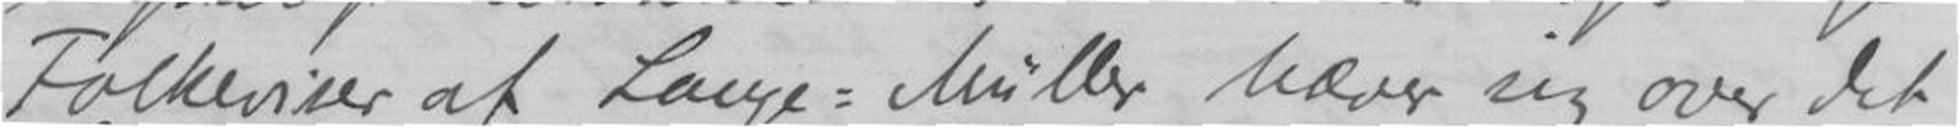Folkeviser af Lange=Müller hæver sig over det
